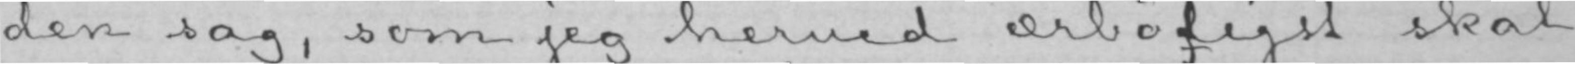den sag, som jeg herved ærbødigst skal
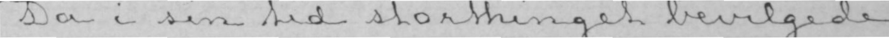Da i sin tid storthinget bevilgede
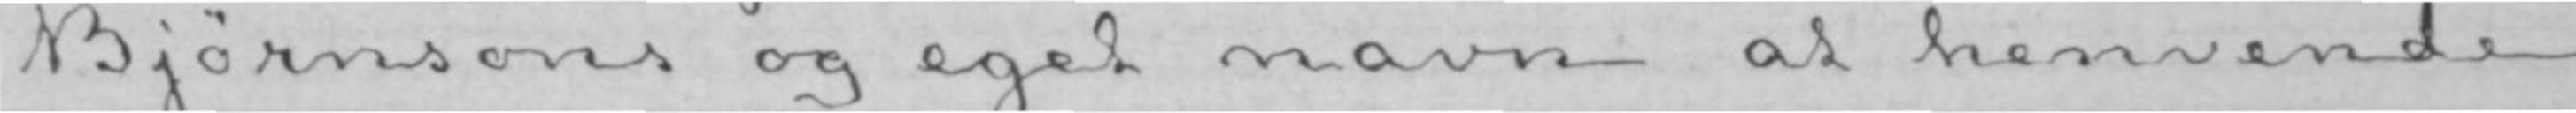Bjørnsons og eget navn at henvende
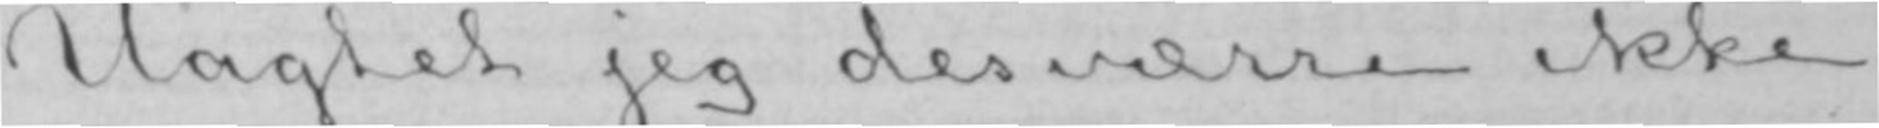Uagtet jeg desværre ikke
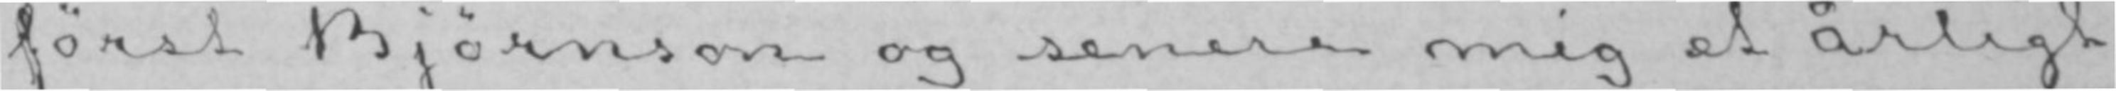først Bjørnson og senere mig et årligt
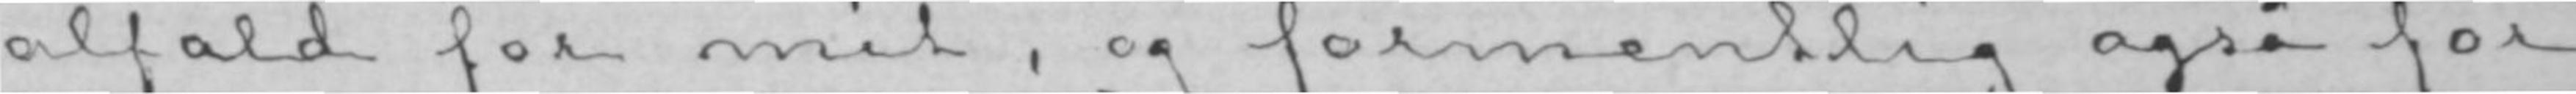alfald for mit, og formentlig også for
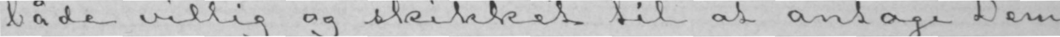både villig og skikket til at antage Dem
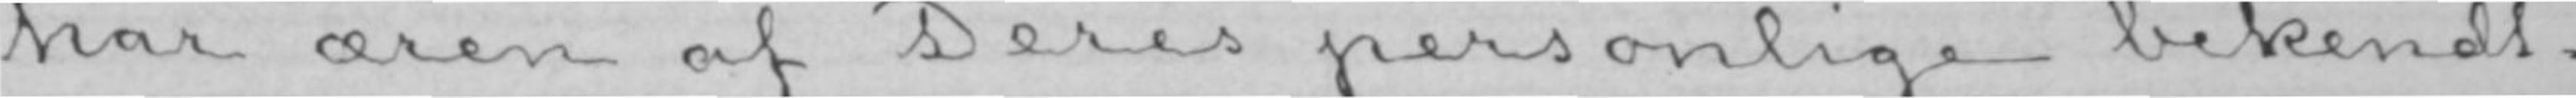har æren af Deres personlige bekendt-
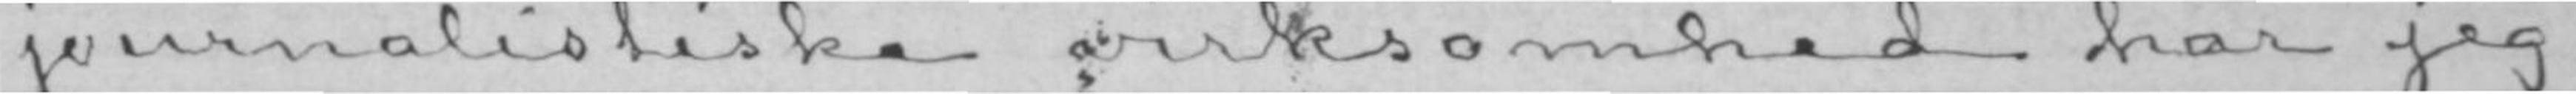journalistiske virksomhed har jeg
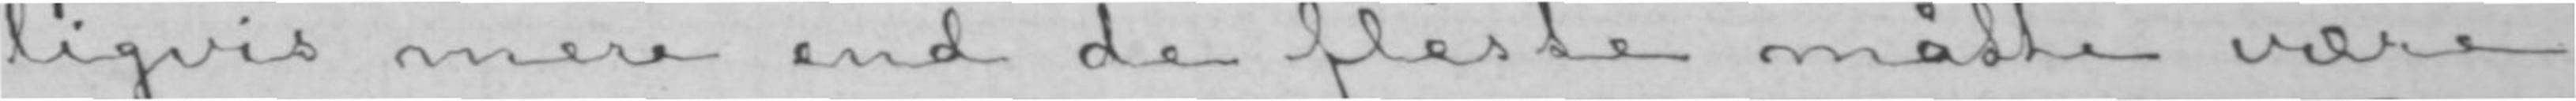ligvis mere end de fleste måtte være
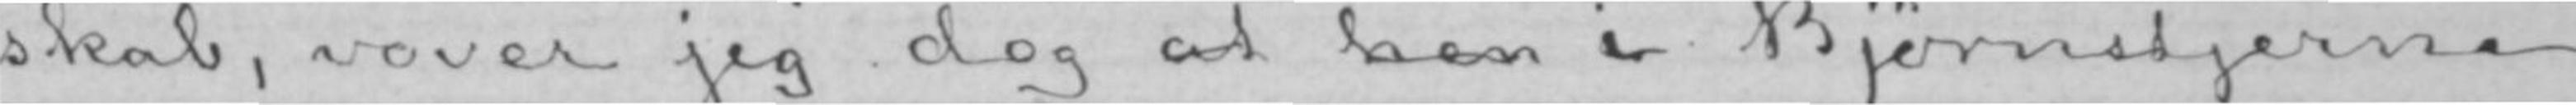skab, vover jeg dog i Bjørnstjerne
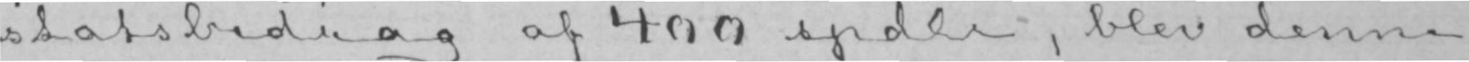statsbidrag af 400 spdlr:, blev denne
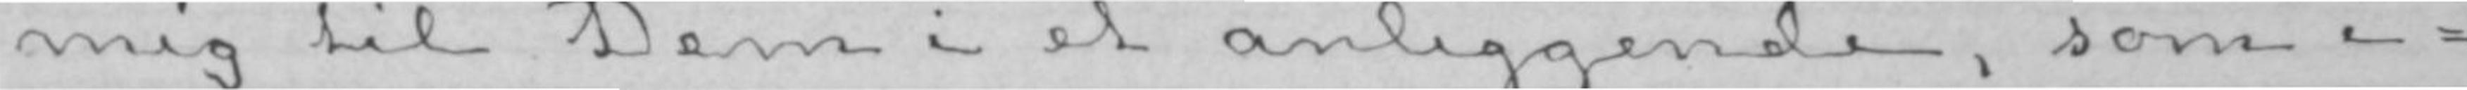mig til Dem i et anliggende, som i-
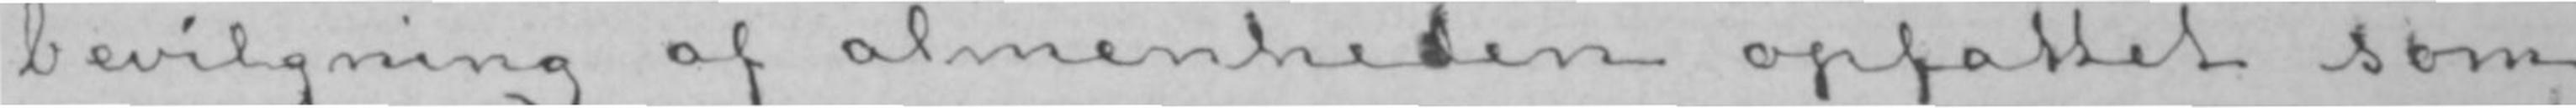bevilgning af almenheden opfattet som en
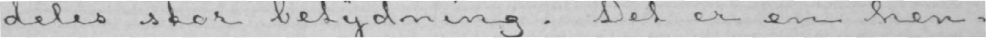deles stor betydning. Det er en hen-
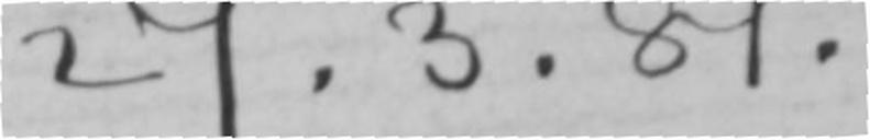27. 3. 81.
